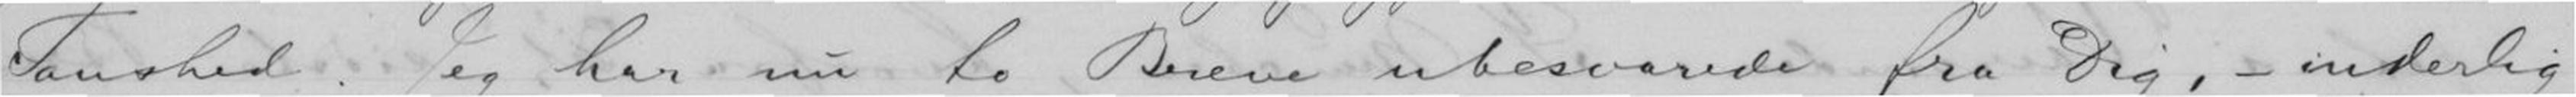Taushed. Jeg har nu to Breve ubesvarede fra Dig, - inderlig
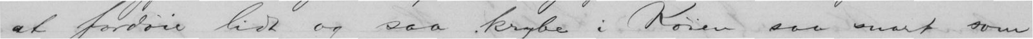at fordrive lidt og saa krybe i Køien saa snart som
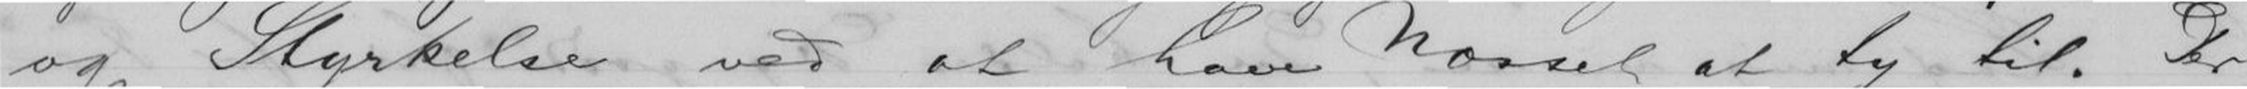og Styrkelse ved at have Næsset at ty til. Der
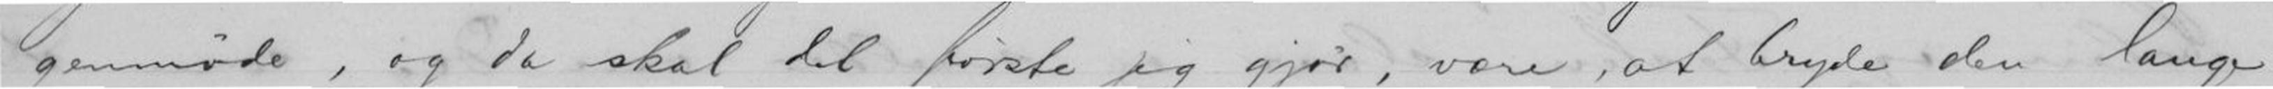genmøde, og da skal det første jeg gjør, være, at bryde den lange
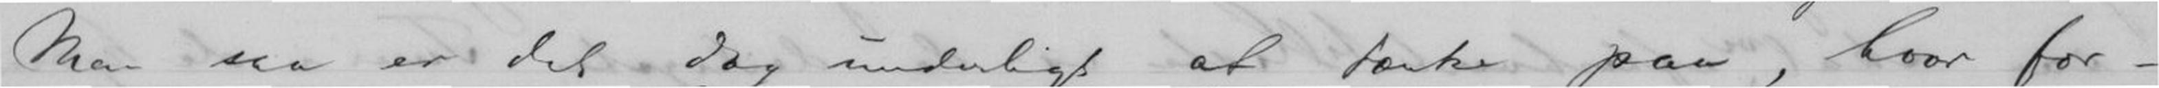Men saa er det dog underligt at tænke paa, hvor for-
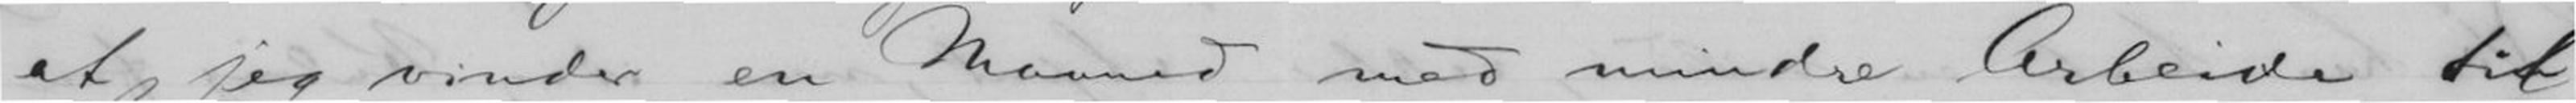at jeg vinder en Maaned med mindre Arbeide til
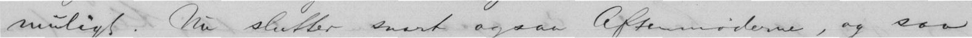muligt. Nu slutter snart ogsaa Aftenmøderne, og saa
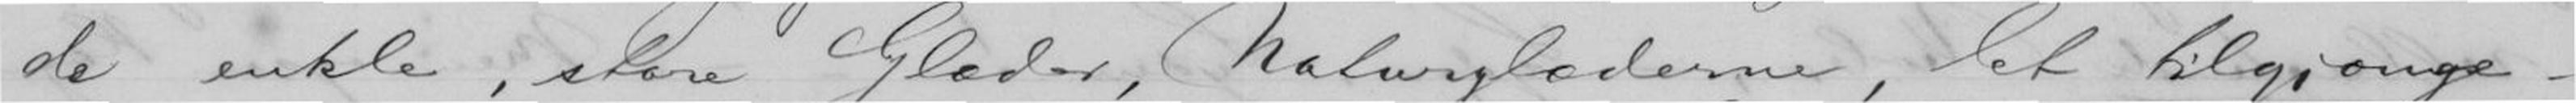de enkle, store Glæder, Naturglæderne, let tilgjænge-
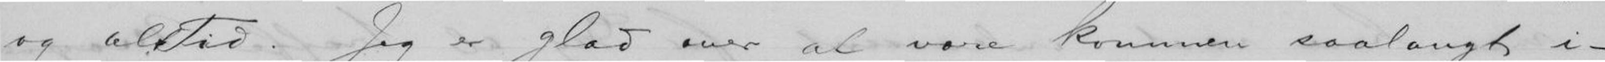og al Tid. Jeg er glad over at være kommen saalangt i-
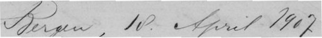Bergen, 18 April 1907.
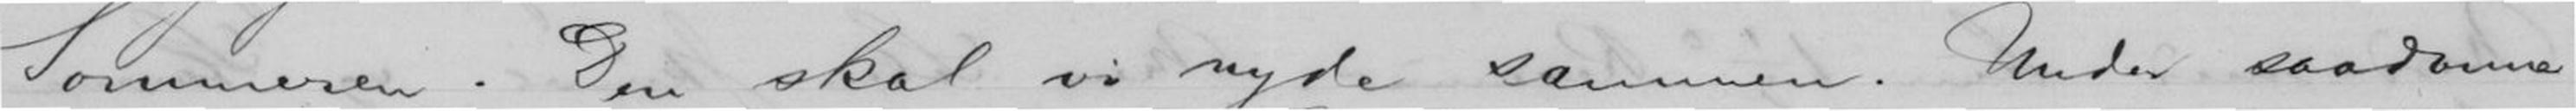Sommeren. Den skal vi nyde sammen. Under saadanne
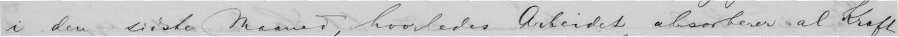i den sidste Maaned, hvorledes Arbeidet absorberer al Kraft
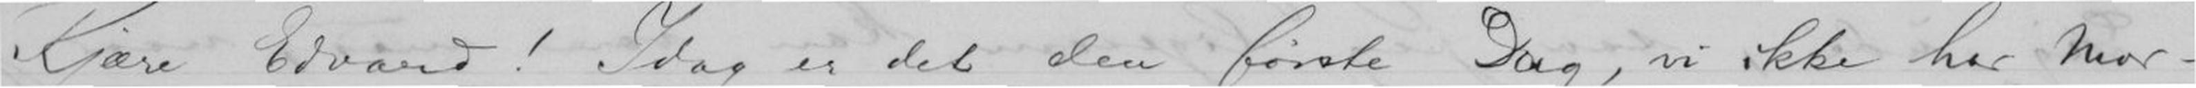Kjære Edvard! Idag er det den første Dag, vi ikke har Mor-
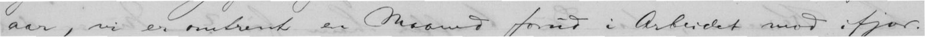aar, vi er omtrent en Maaned forud i Arbeidet mod ifjor.
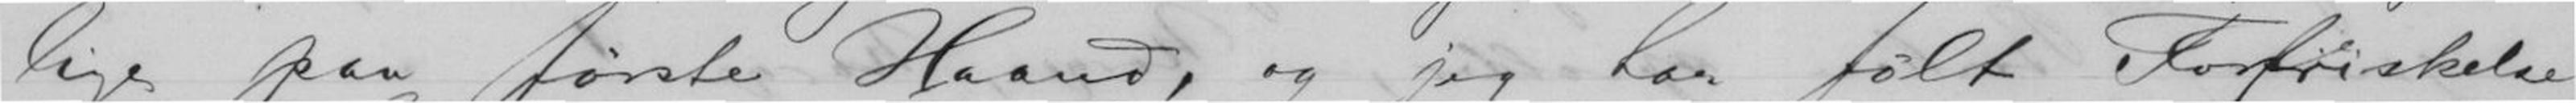lige paa første Haand, og jeg har følt Forfriskelse
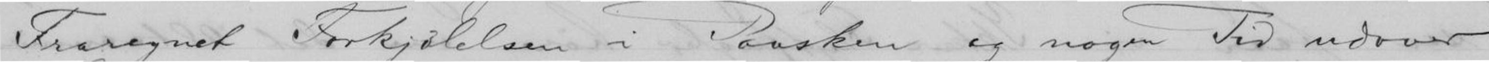Fraregnet Forkjølelsen i Paasken og nogen Tid udover
